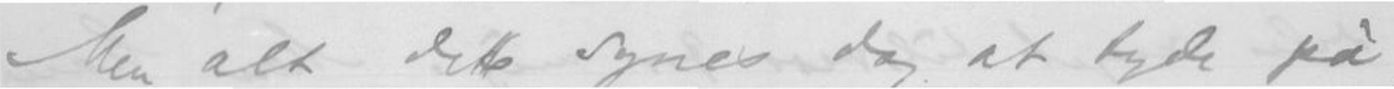Men alt dette synes dog at tyde på,
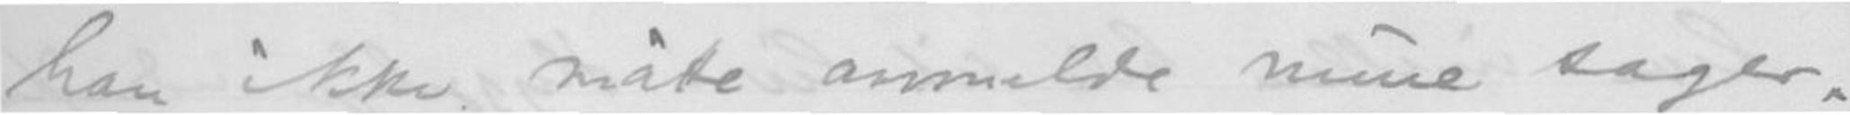han ikke måtte anmelde mine sager.
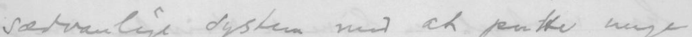sædvanlige system med at putte unge
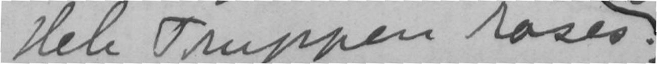Hele Truppen roses
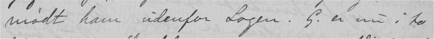mødt ham udenfor Logen. G. er nu i to
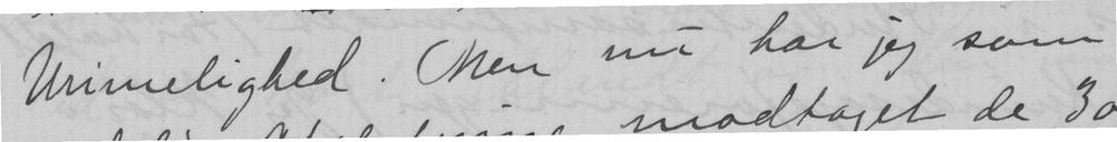Urimelighed. Men nu har jeg som
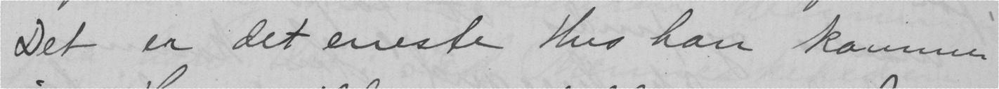Det er det eneste Hus han kommer
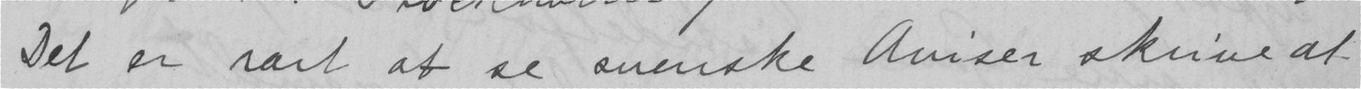Det er rart at se svenske aviser skrive at
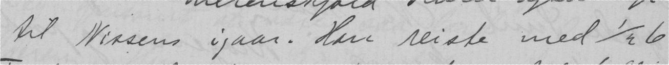til Nissens igaar. Han reiste med 1/2 6
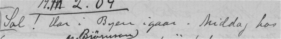Sol! Var i Byen igaar. Middag hos
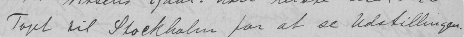Toget til Stockholm for at se Udstillingen.
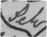selv
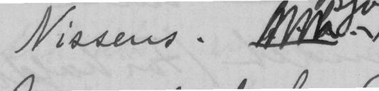Nissens.
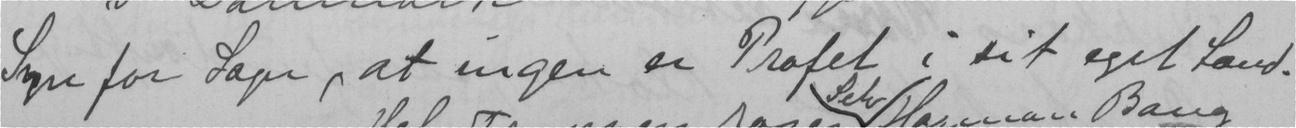Syn for Sagn, at ingen er Profet i sit eget Land.
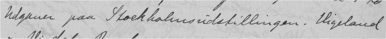Udgaver paa Stockholmsudstillingen. Vigeland
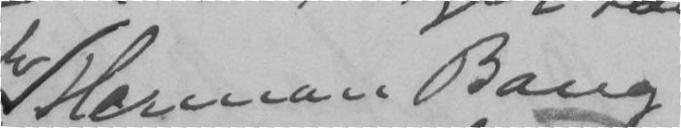Herman Bang
